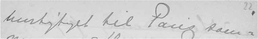hurtigtoget til Paris sam-
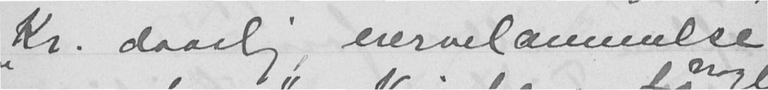"Kr. daarlig, nervelammelse
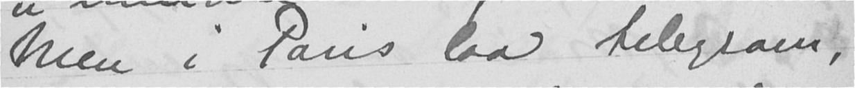Men i Paris laa telegram,
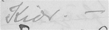Kiær. -
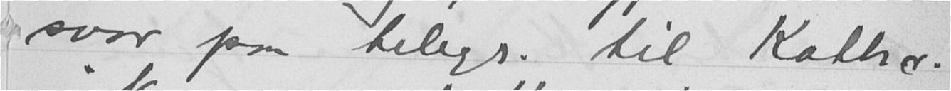svar paa telegr. til Kathr.
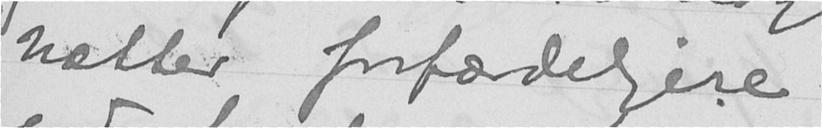nætter forfædeligere
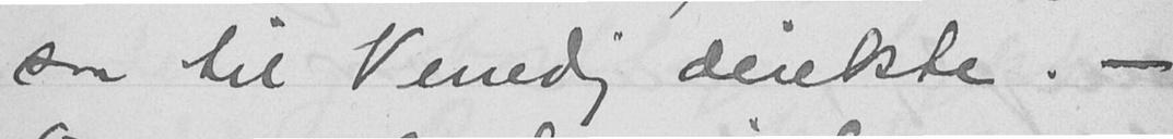saa til Venedig direkte. -
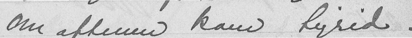Om aftenen kom Sigrid.
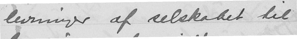levninger af selskabet til
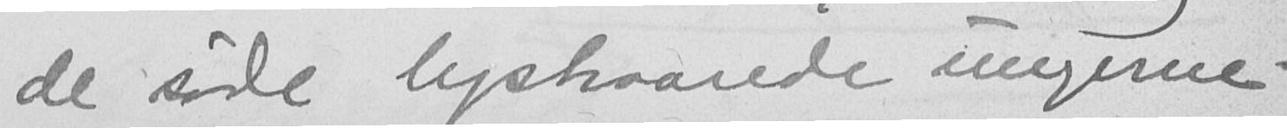de søde lyshaarede ungerne.
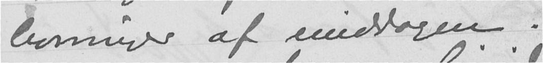levninger af middagen.
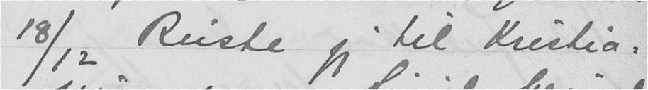18/12 Reiste jeg til Kristia-
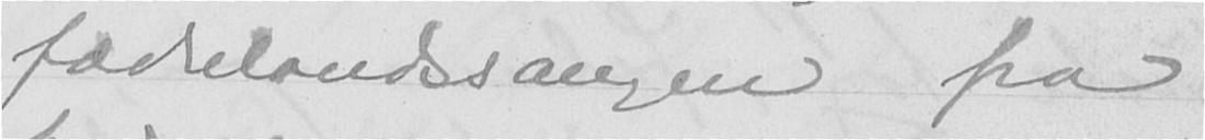fædrelandssangen fra
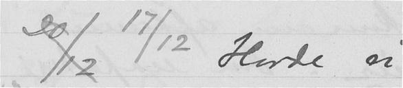20/12 17/12 Havde vi
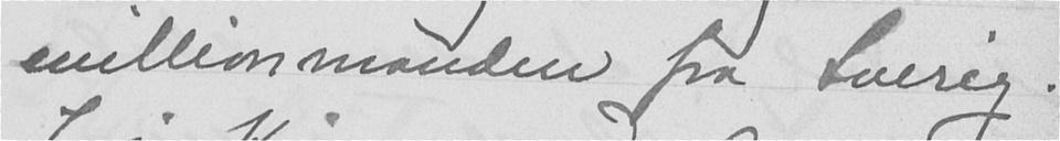millionmanden fra Sverig.
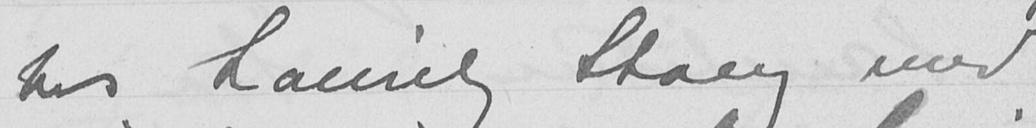hos Lauritz Stang med
